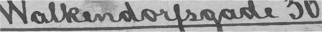Walkendorfsgade 30,
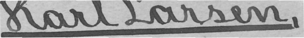Karl Larsen,
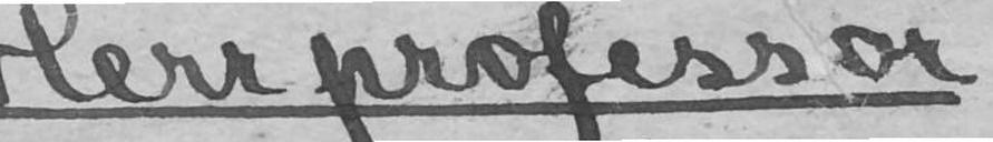Herr professor
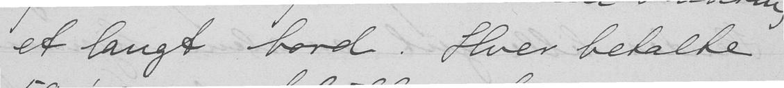et langt bord. Hver betalte
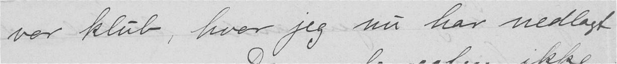vor klub, hvor jeg nu har nedlagt
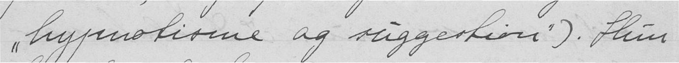hypnotisme og suggestion"). Hun
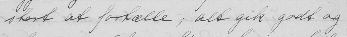stort at fortælle; alt gik godt og
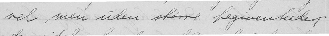vel men uden større begivenheder;
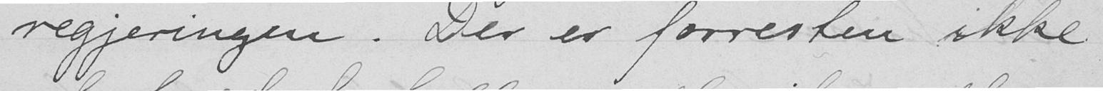regjeringen. Der er forresten ikke
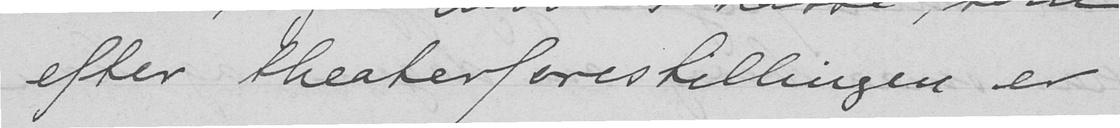efter theaterforestillingen er
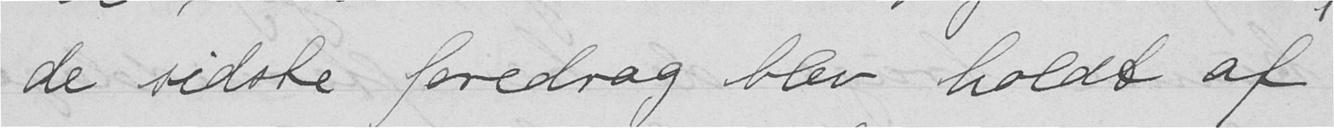de sidste foredrag blev holdt af
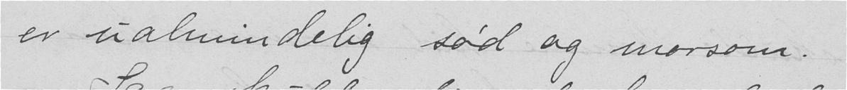er ualmindelig sød og morsom.
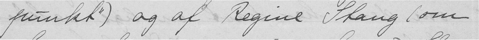punkt") og af Regine Stang (om
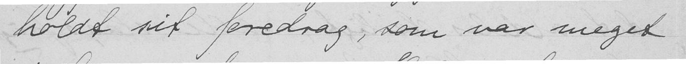holdt sit foredrag, som var meget
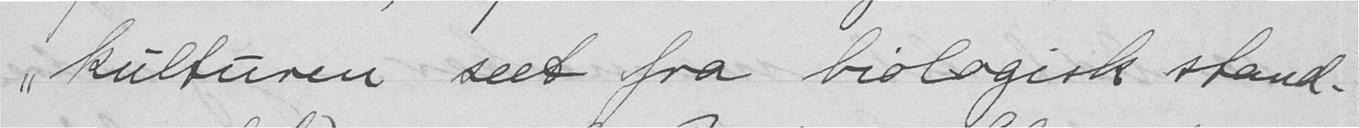kulturen seet fra biologisk stand-
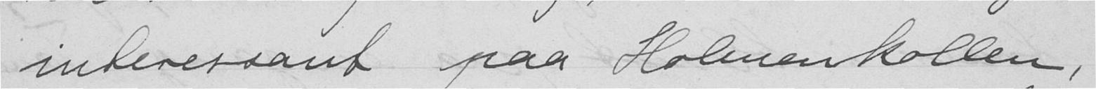interessant paa Holmenkollen,
